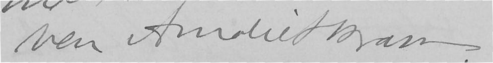ven Amalie Skram.
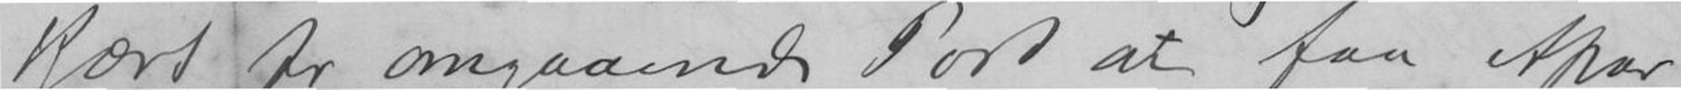kjært pr omgaaende Post at faa etpar
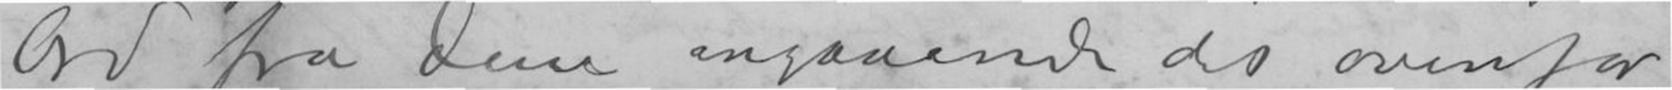Ord fra Dem angaaende de ovenfor
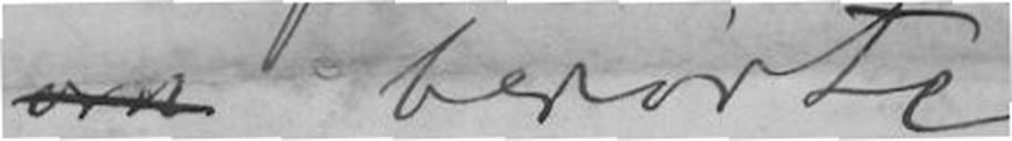om berørte.
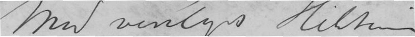Med venliges Hilsen
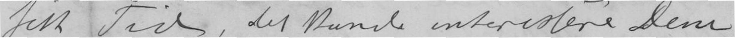fik Tid, det kunde interesere Dem
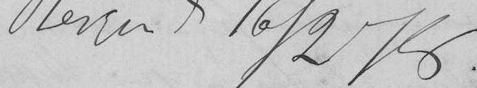Bergen d. 16/12 78.
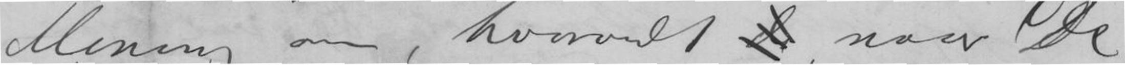Mening om, hvorvidt, d naar De
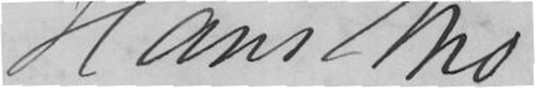Hans Mo
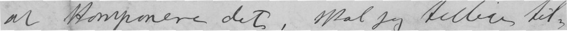at komponere det, skal jeg tillige til-
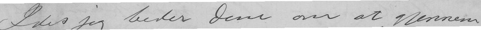Idet jeg beder Dem om at gjennem-
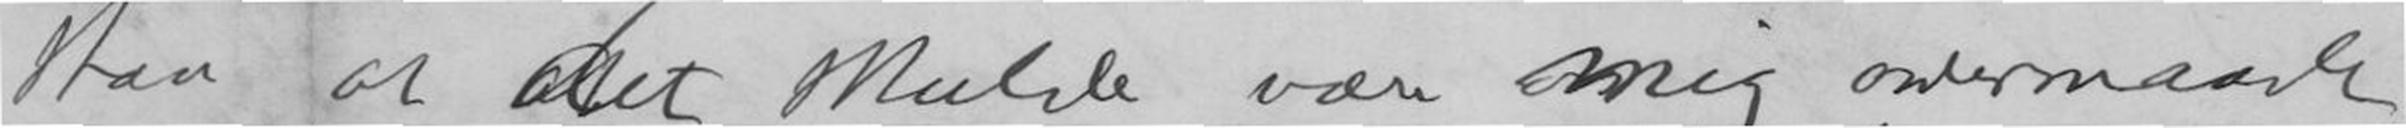staa at det skulde være mig overmaade
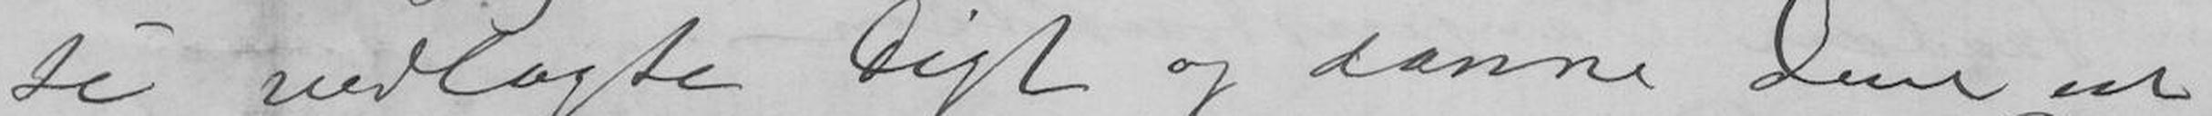sé vedlagte Digt og danne Dem en
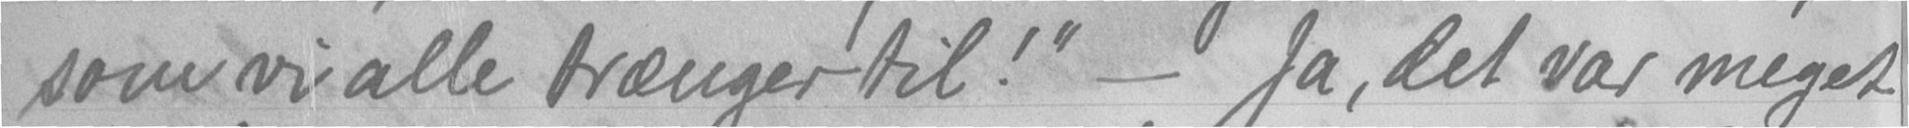som vi alle trænger til!" - Ja, det var meget
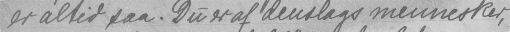er altid saa. Du er af denslags mennesker,
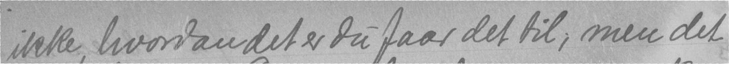ikke, hvordan det er du faar det til, men det
